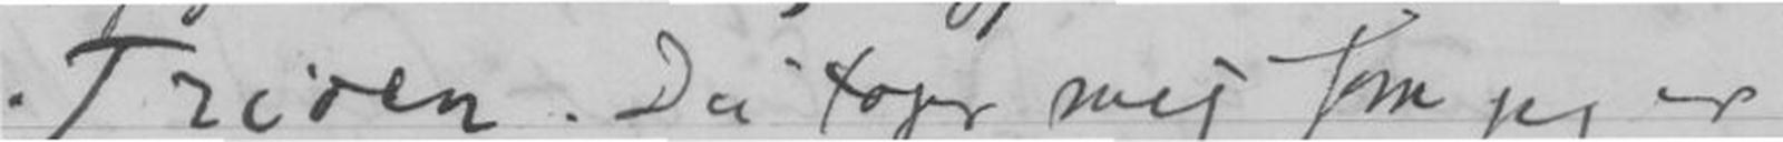Trioen. Du tager mig som jeg er
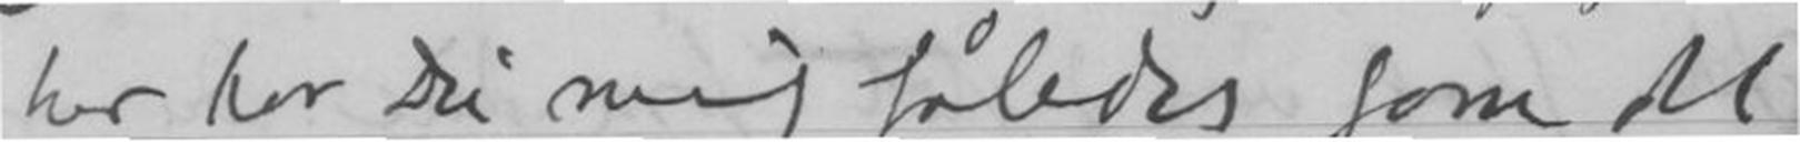her har Du mig således som det
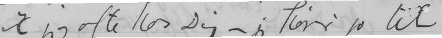er jeg ofte hos Dig - jeg Hører jo lit
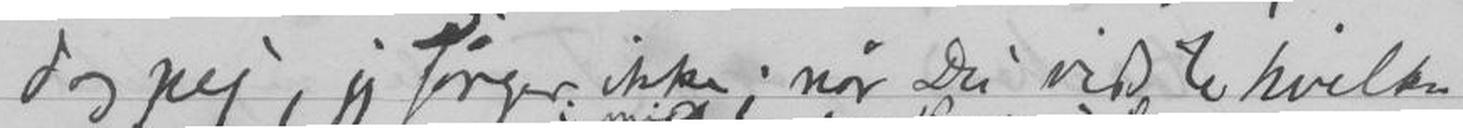dog nej, jeg sørger ikke; når Du vidste hvilken
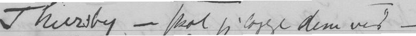Thursby - skal jeg lægge dem ved -
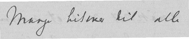Mange hilsener til alle
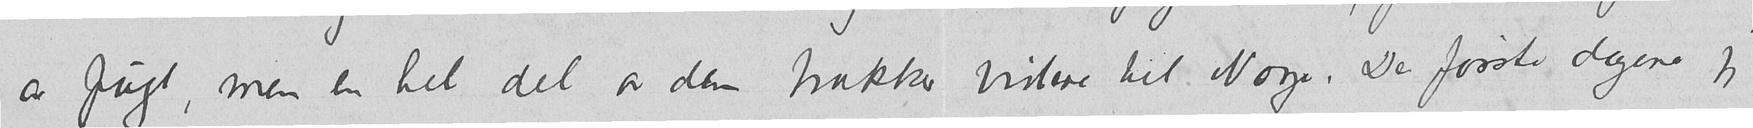av fugl, men en hel del av dem trækker videre til Norge. De første dagene jeg
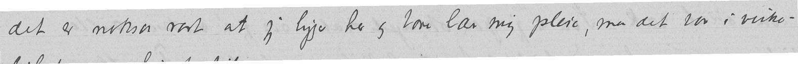det er noksaa rart at jeg ligger her og bare lar mig pleie, men det var i virke-
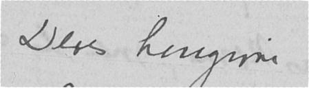Deres hengivne
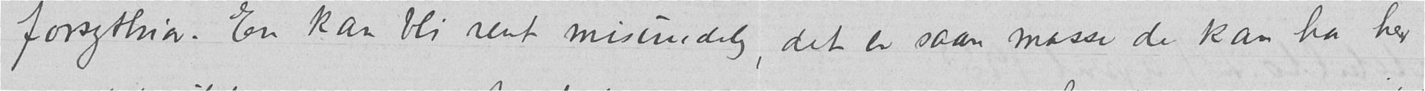forsythia. En kan bli rent misundelig, det er saan masse de kan ha her
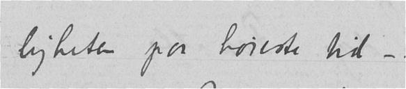ligheten paa høieste tid –.
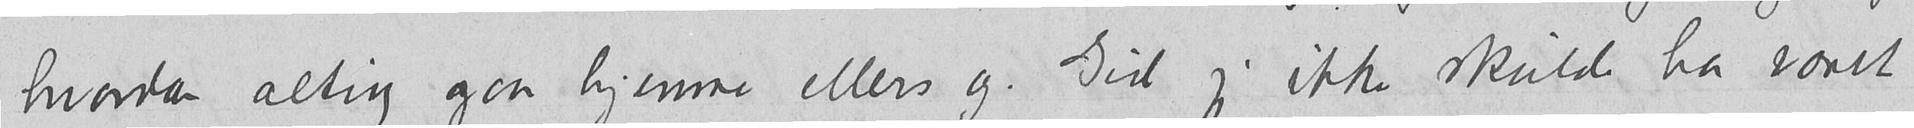hvordan alting gaar hjemme ellers og. Gid jeg ikke skulde ha været
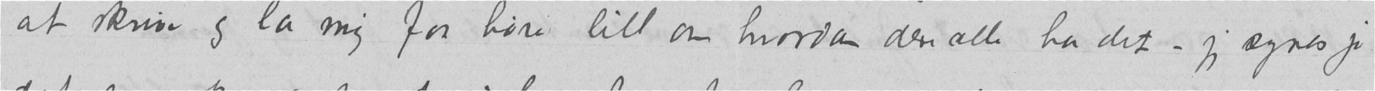at skrive og la mig faa høre litt om hvordan dere alle har det – jeg synes jo
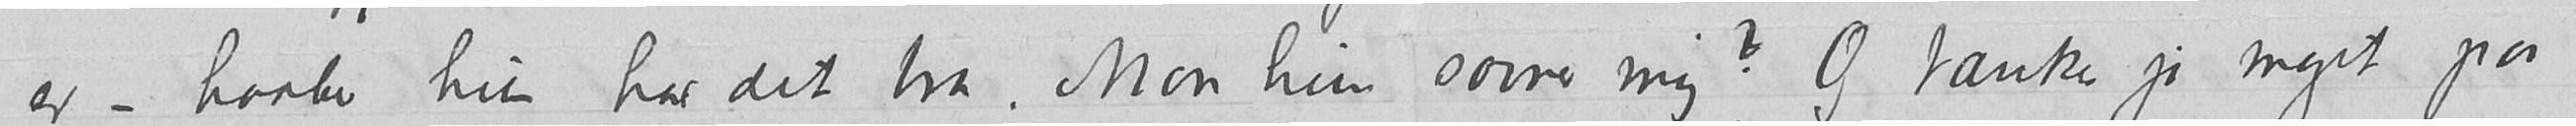er – haaber hun har det bra. Mon hun savner mig? Og tænker jo meget paa
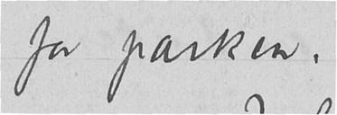for parken.
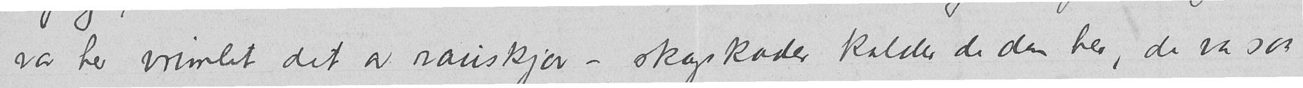var her vrimlet det av rauskjør – skogskader kalder de dem her, de var saa
